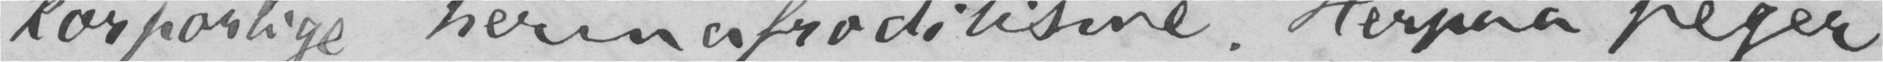korporlige hermafroditisme. Herpaa peger
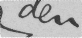den
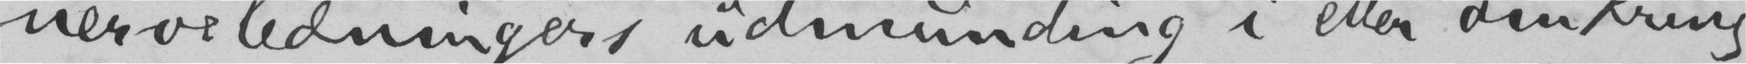nerveledningers udmunding i eller omkring
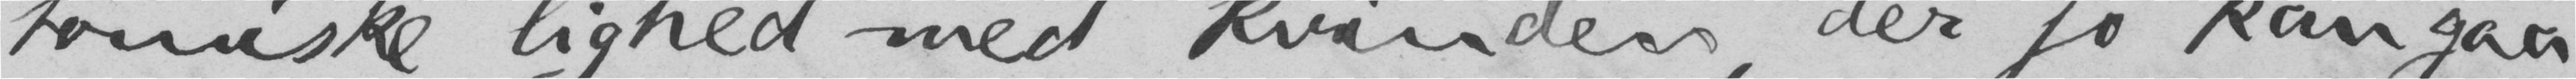tomiske lighed med kvinden, der jo kan gaa
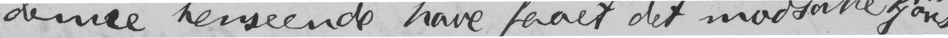denne henseende have faaet det modsatte kjøns
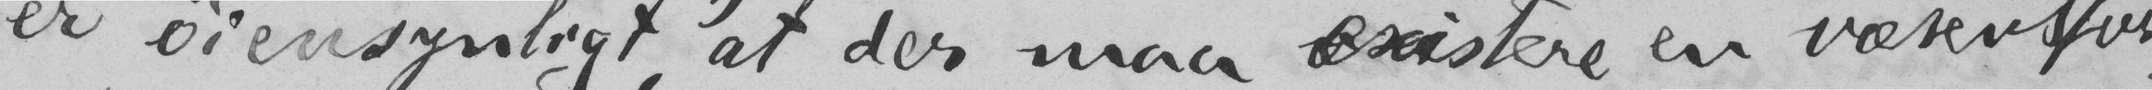er øiensynligt, 1) at der maa existere en væsensfor-
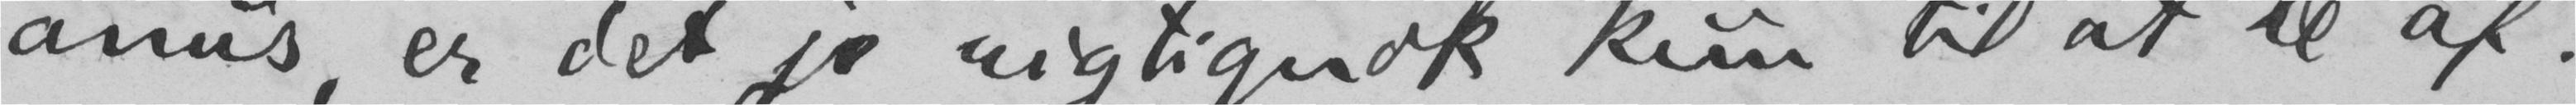anus, er det jo rigtignok kun at le af.
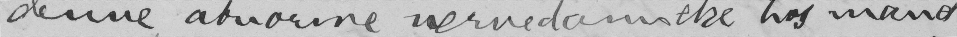denne abnorme nervedannelse hos mand
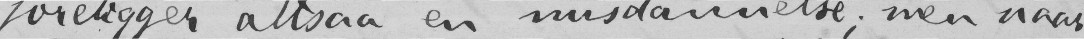foreligger altsaa en misdannelse; men naar
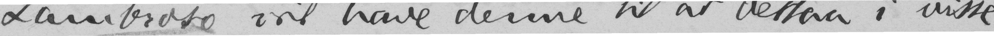Lombroso vil have denne til at bestaaa i visse
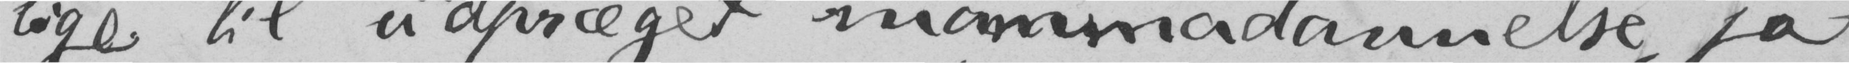lige til udpræget mammadannelse, ja
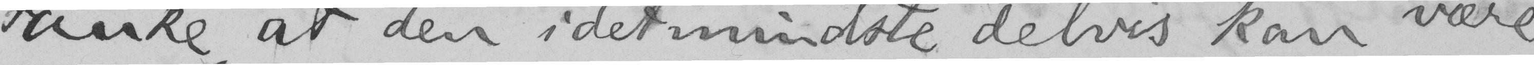tanke, at den idetmindste delvis kan være
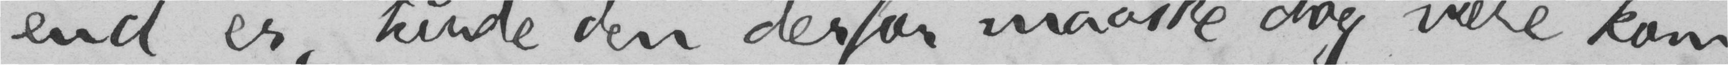end er, turde den derfor maaske dog være kom-
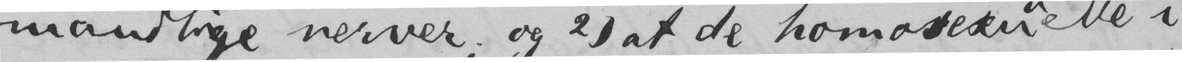mandlige nerver, og 2) at de homosexuelle i
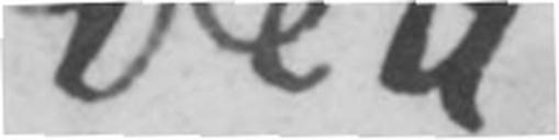ved
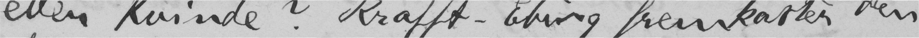eller kvinde? Krafft-Ebing fremkaster den
